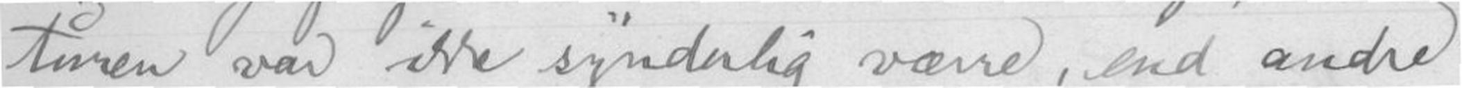turen var ikke synderlig værre, end andre
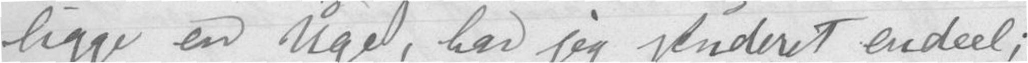ligge en Uge, har jeg studeret endeel;
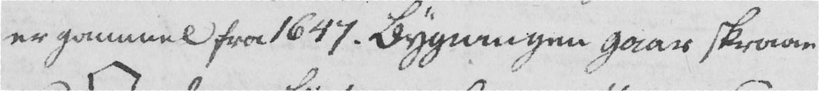er gammel fra 1647. Bygningen gaar skraae
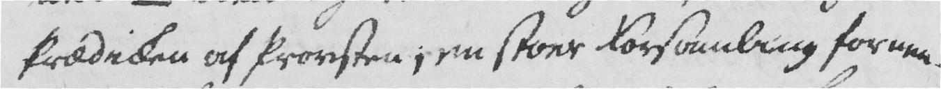Prædiken af Provsten; en stoer forsamling fornem-
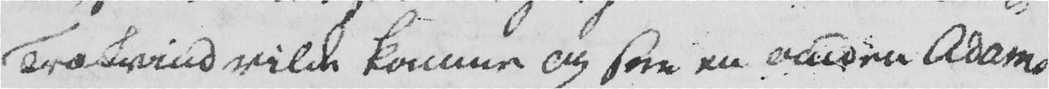Trækvind vilde komme og see en anden Adams
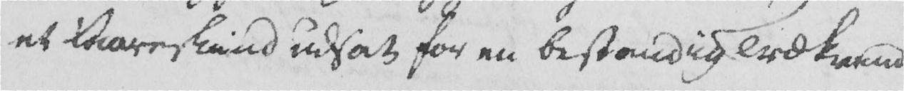et Faareskind udsat for en bestandig Trækvind.
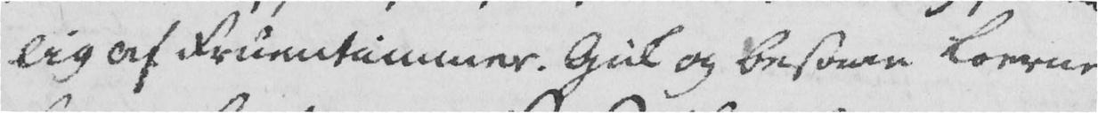lig af Fruentimmer. Gik og besaae Lovene
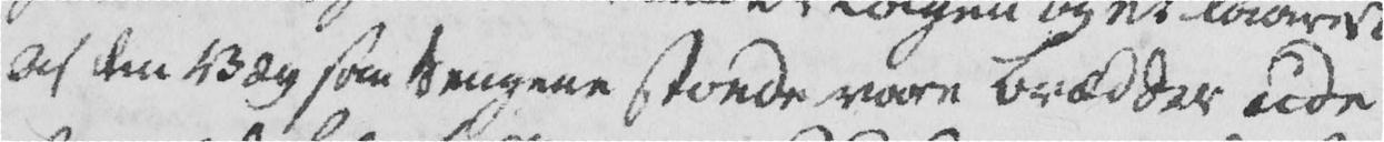af den Væg som Sengene stoede vare Brædder ude
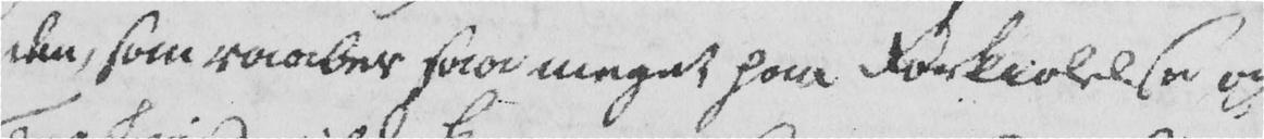den, som raaber saa meget paa Forkiølelse og
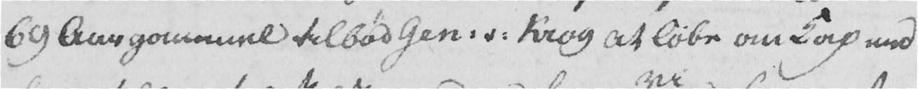69 Aar gammel tilbød Gen. v. Krog at løbe om Kap med
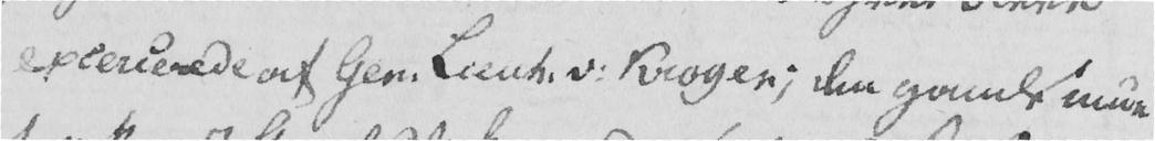excercerede af Gen. Lieut. v. Krogen; den gamle mun-
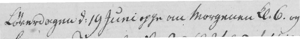Løverdagen d. 19 Juni oppe om Morgenen kl. 6. og
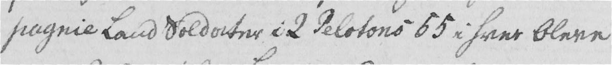pagnie Land Soldater i 2 Pelotons 55 i hver bleve
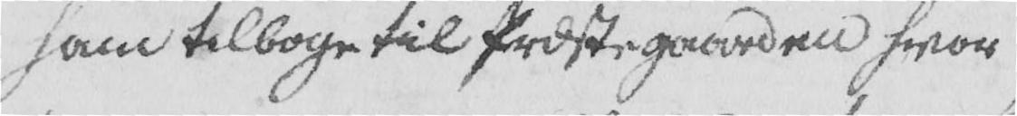ham tilbage til Præstegaarden hvor
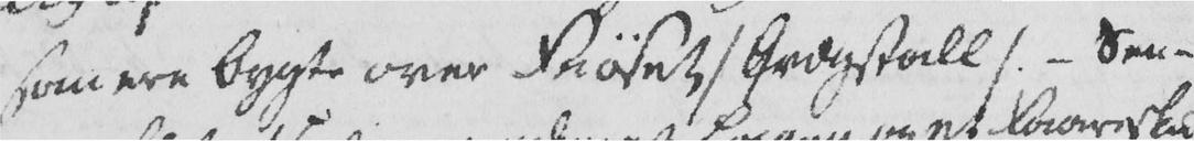som ere bygte over Fiøset / Qvægstall /. - Sen-
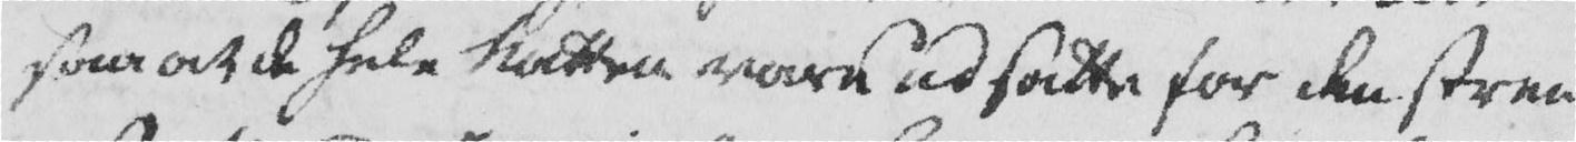saa at de hele Natten vare udsatte for den stren-
